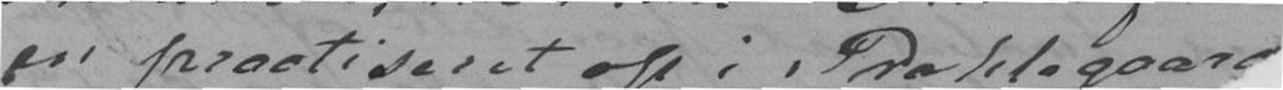en practiseret op i Prahlegaard
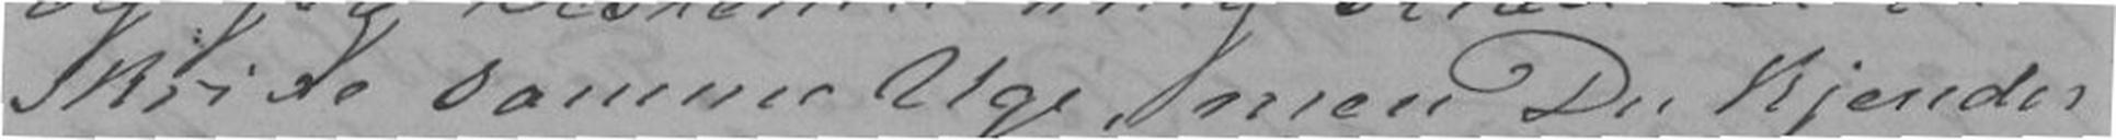skrive samme Uge, men Du kjender
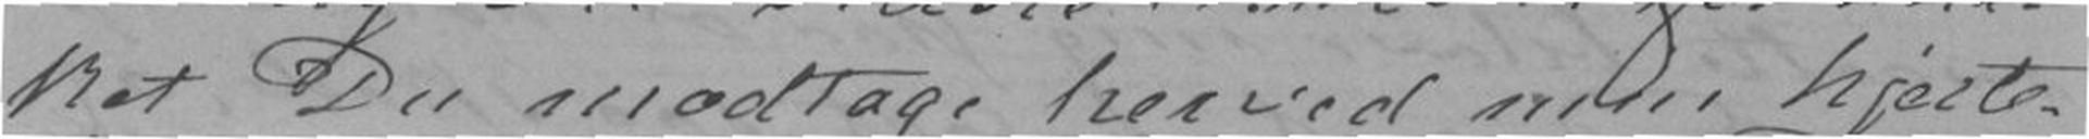ket Du modtage herved min hjerte-
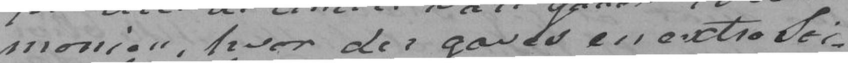monien, hvor der gaves en extra Soi-
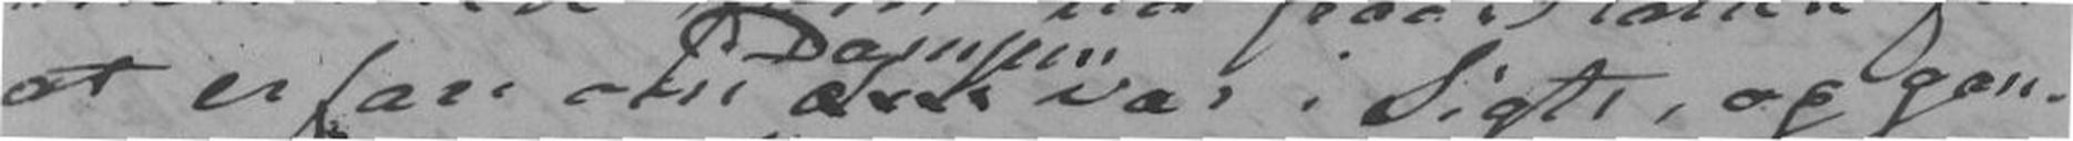at erfare om Dampen var i Sigte og gan-
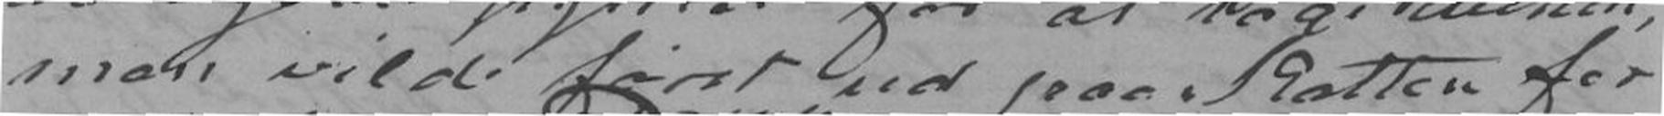men vilde først ud paa Skolten for
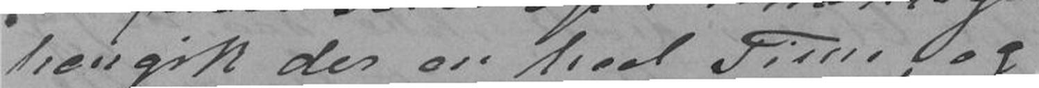hengik der en heel Time, og
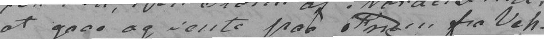at gaae og vente paa Fruen fra Vah
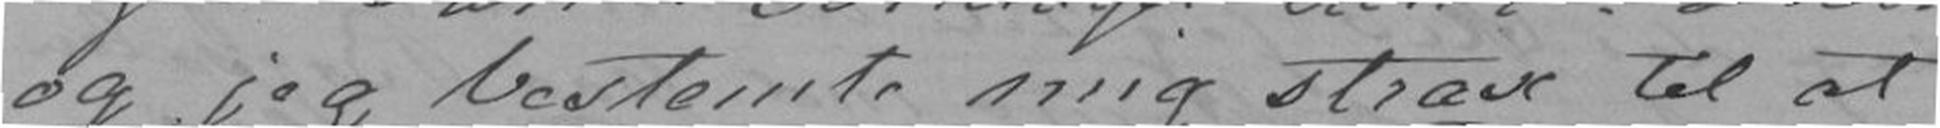og jeg bestemte mig strax til at
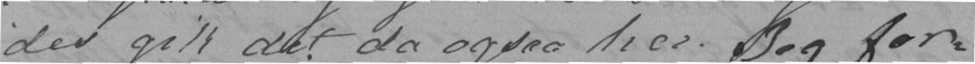des gik det da ogsaa her. Jeg for-
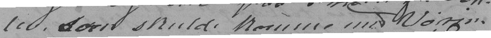len, som skulde komme med Vørin-
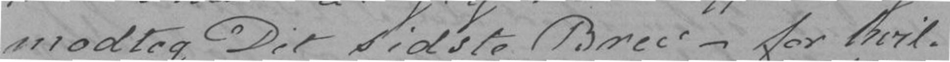modtog Dit sidste Brev - for hvil
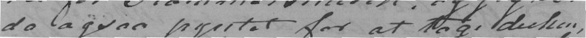da ogsaa pyntet for at tage derhen,
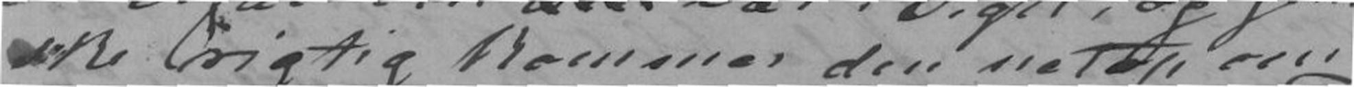ske rigtig kommer den netop, om
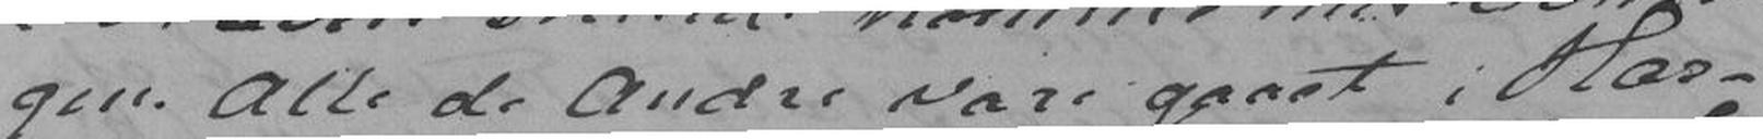gen. Alle de Andre vare gaaet i Har-
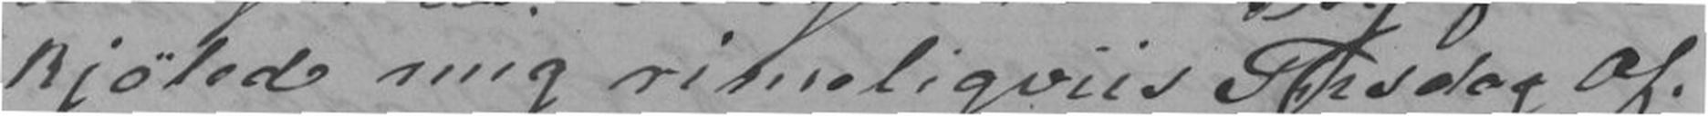kjølede mig rimeligvis Tirsdag af-
